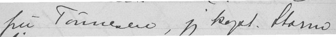fru Tønnesen, jeg kapt. Storm
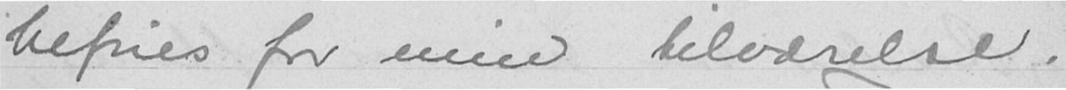befries for min tilværelse.
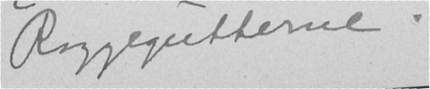Roggegutterne.
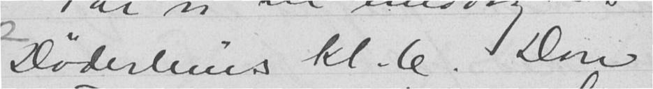Døderleins kl. 6. Don
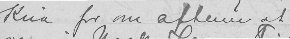Kria for om aftenen at
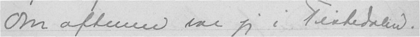Om aftenen var jeg i Tistedalen.
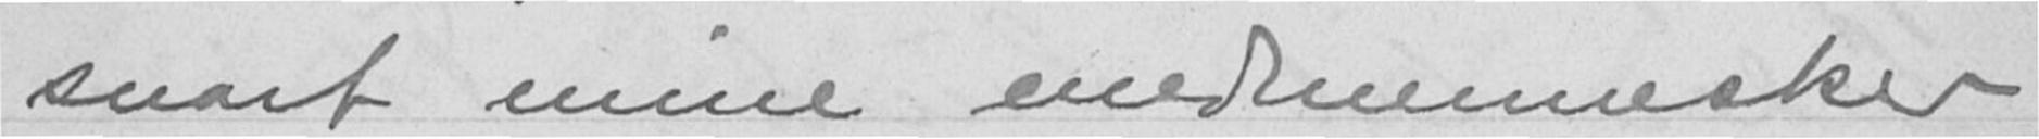snart mine medmennesker
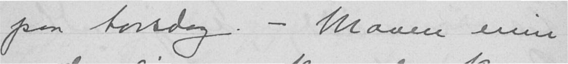paa torsdag. - Maven min
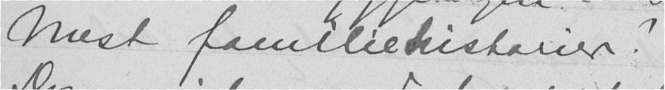Mest familiehistorier?
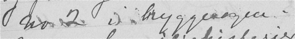no 2 i bryggesagen.
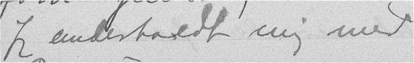Jeg underholdt mig med
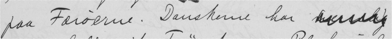paa Færøerne. Danskerne har
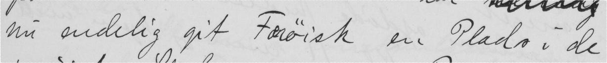nu endelig git Færøisk en Plads i de
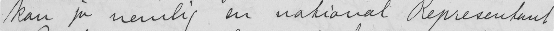kan jo nemlig en national Representant
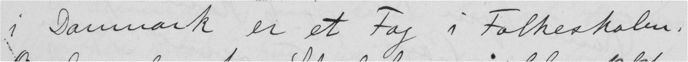i Danmark er et Fag i Folkeskolen.
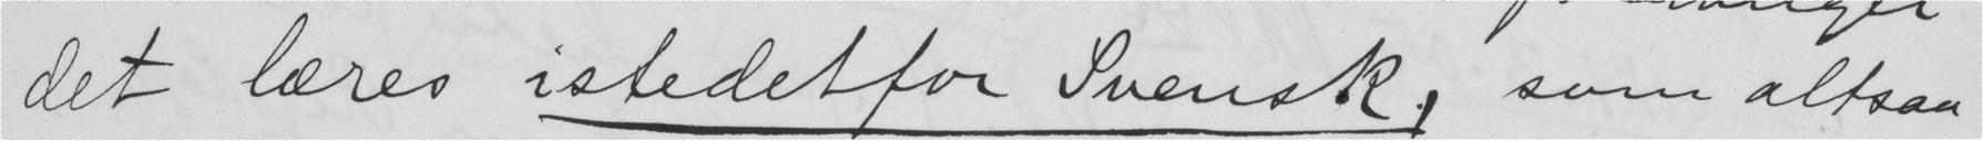det læres istedetfor Svensk, som altsaa
